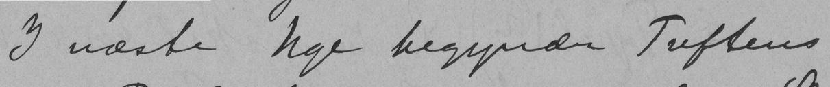I næste Uge begyner Tuftens
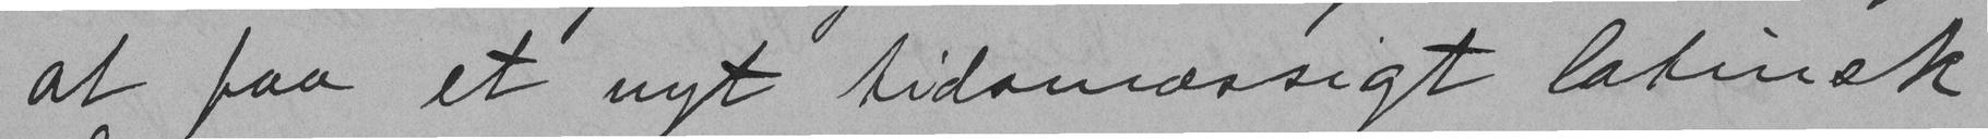at faa et nyt tidsmæssigt latisk
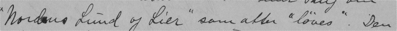"Nordens Lund og Lier" som atter "løves". Den
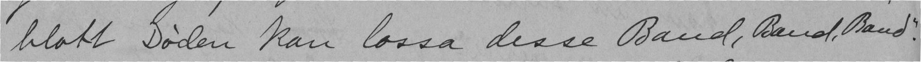blott Døden kan lossa desse Band, Band, Band."
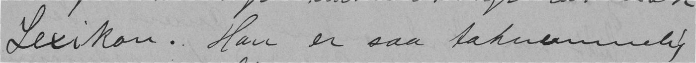Lexikon. Han er saa taknemmelig
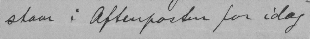staar i Aftenposten for idag
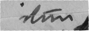hun
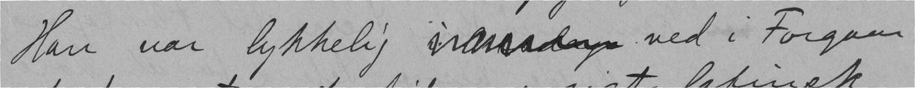Han var lykkelig  Mandags ved i Forgaar
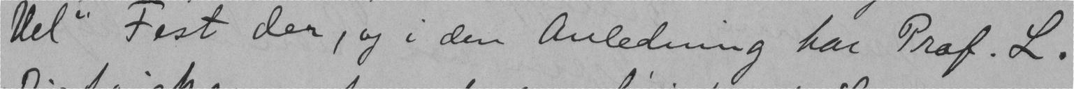Vel" Fest der, og i den Anledning har Prof. L.
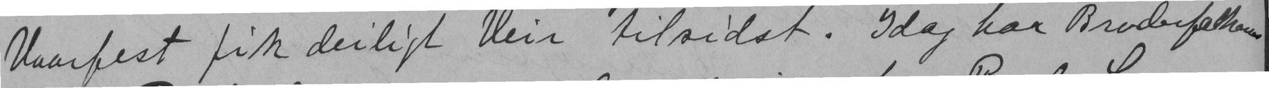Vaarfest fik deiligt Veir tilsidst. Idag har" Broderfolkernes
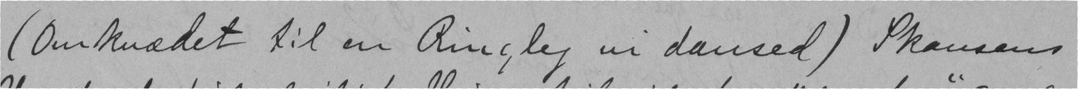(Omkvædet til en Ringleg vi dansed) Skansens
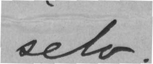selv.
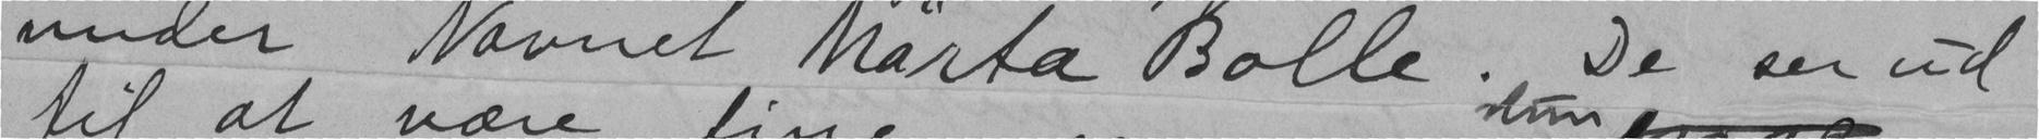under Navnet Märta Bolle. De ser ud
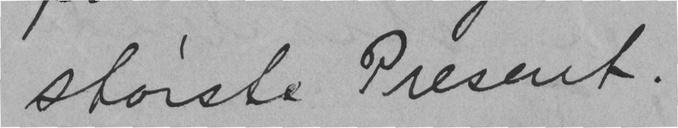største Present.
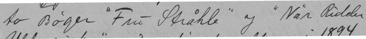to Bøger "Fru Stråhle" og "När Ridder
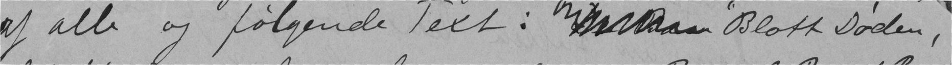af alle og følgende Text:  "Blott Døden,
